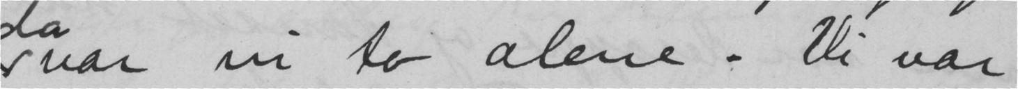var vi to alene. Vi var
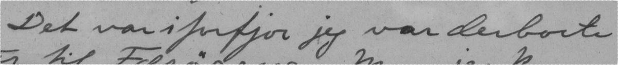Det var i forfjor jeg var derborte
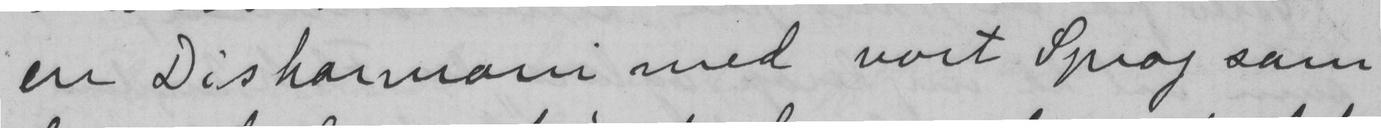en Disharmoni med vort Sprog som
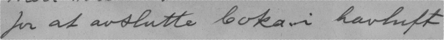for at avslutte boka i havluft
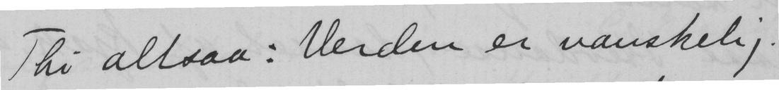Thi altsaa: Verden er vanskelig.
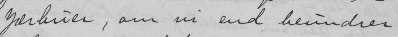Jærbuer, om vi end beundrer
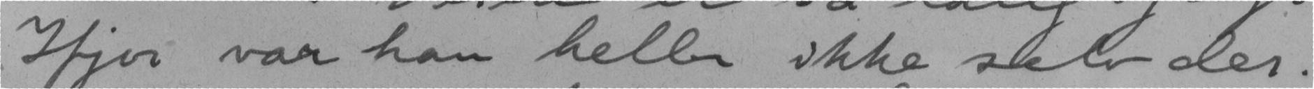Ifjor var han heller ikke slelv der.
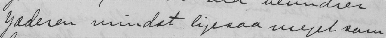Jæderen mindst ligesaa meget som
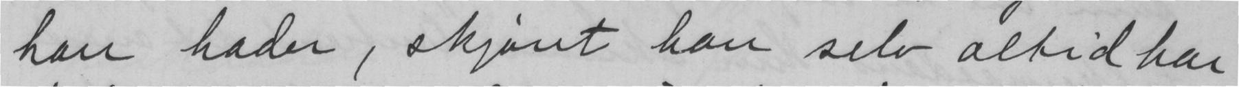han hader, skjønt han selv altid har
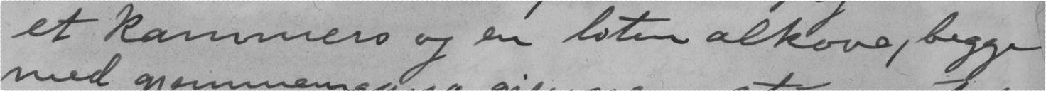et kammers og en liten alkove, begge
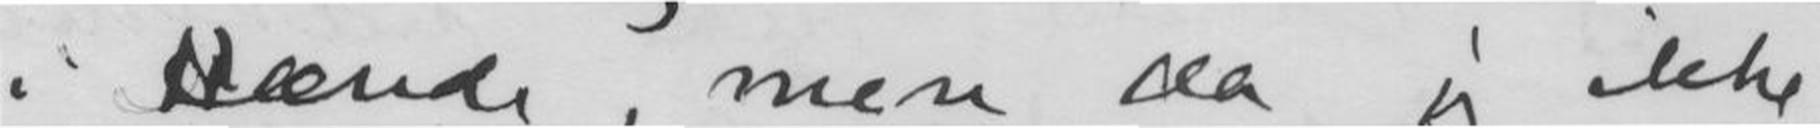i Hænde, men da jeg ikke
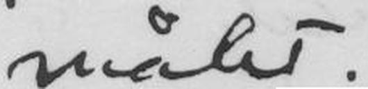målet.
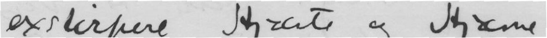exs..rpere Hjærte og Hjærne
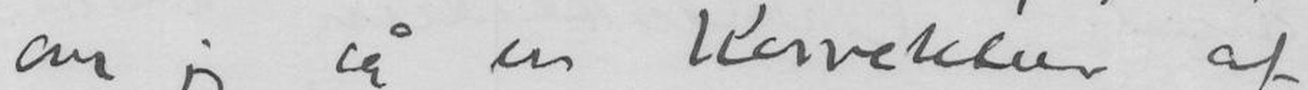om jeg så en Korrektur af
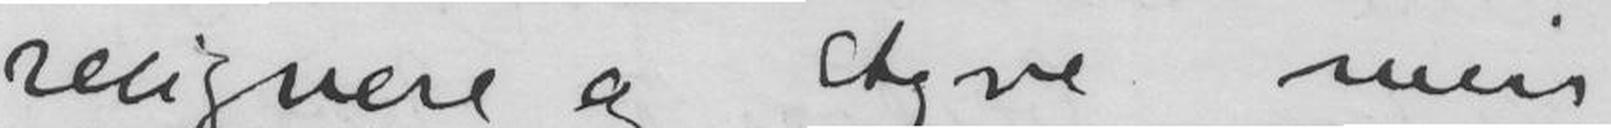resignere og styre min
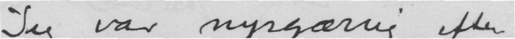Jeg var nysgærrig efter
